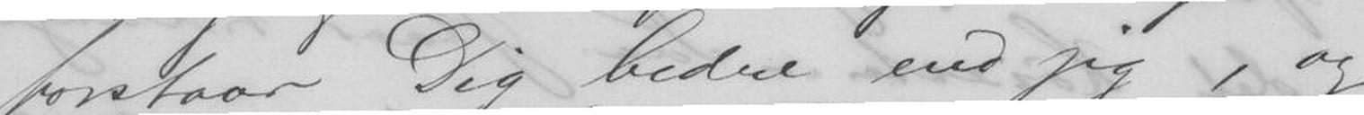forstaar Dig bedre end jeg, og
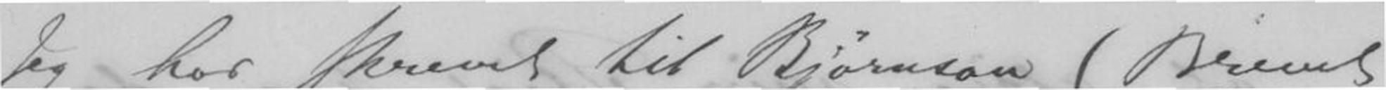Jeg har skrevet til Bjørnson (Brevet
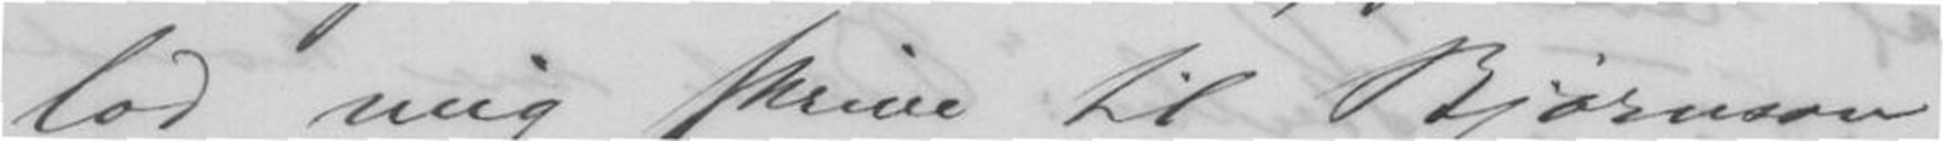lod mig skrive til Bjørnson,
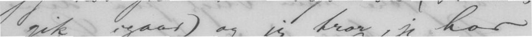gik igaar) og jeg tror, jeg har
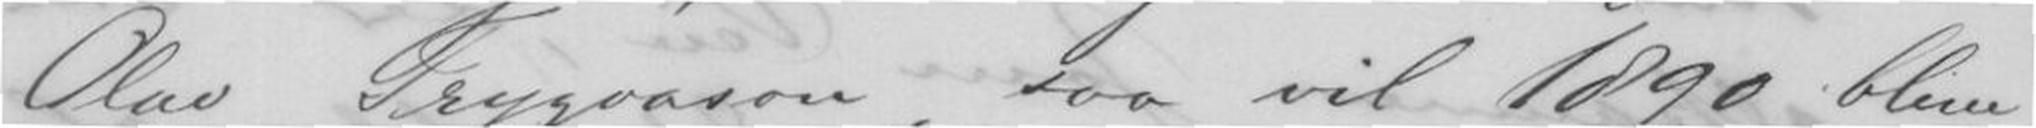Olav Trygvason, saa vil 1890 blive
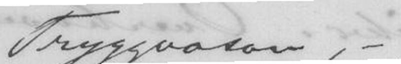Tryggvason, -
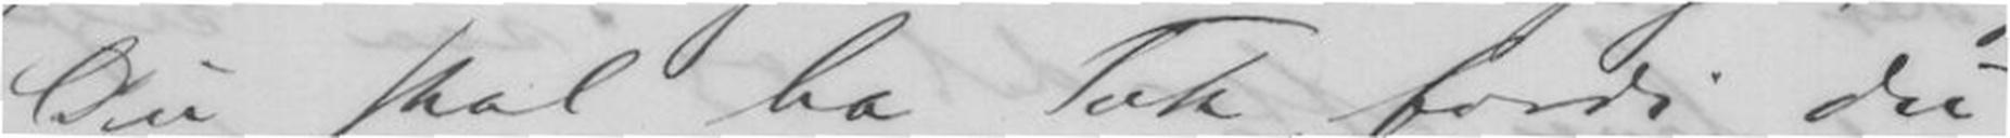Du skal ha Tak, fordi du
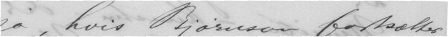--- ja, hvis Bjørnson fortsætter
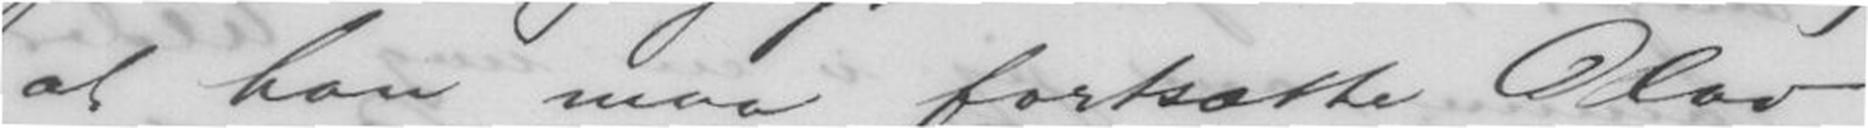at han maa fortsætte Olav
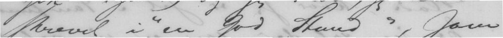skrevet i "en god Stund", som
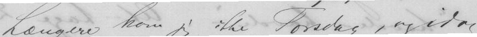Længere kom jeg ikke Torsdag, og idag
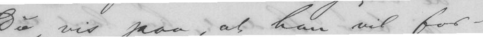Du vis paa, at han vil for-
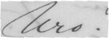Uro.
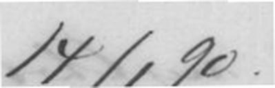14/1 90
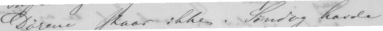Dørene staar ikke. Søndag havde
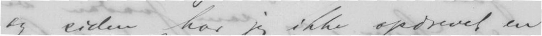og siden har jeg ikke opdrevet en
